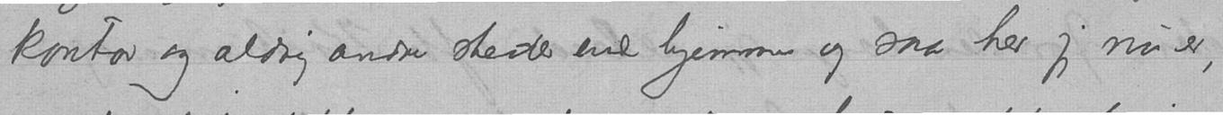kontor og aldrig andre steder end hjemme og saa her jeg nu er,
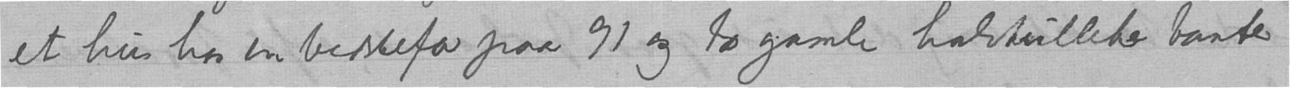et hus hos en bedstefar paa 91 og to gamle halvtullete tanter
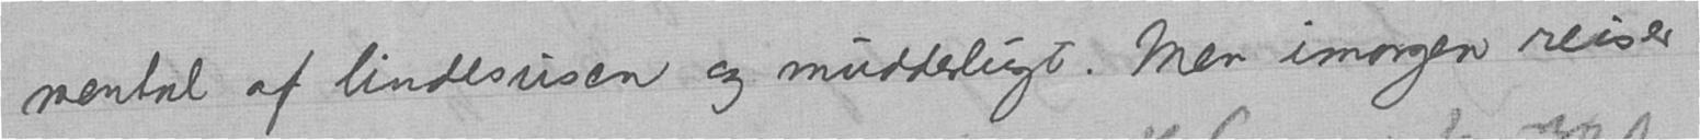mental af lindesusen og mudderlugt. Men imorgen reiser
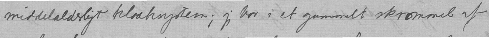middelalderligt kloaksystem; jeg bor i et gammelt skrammel af
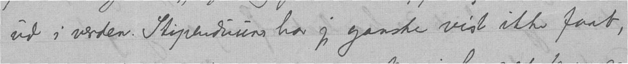ud i verden. Stipendiums har jeg ganske vist ikke faat,
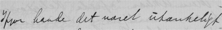Ifjor havde det været utænkeligt
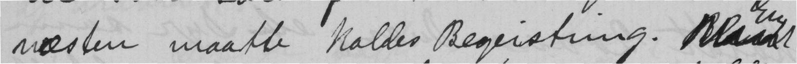næsten maatte kaldes Begeistring.
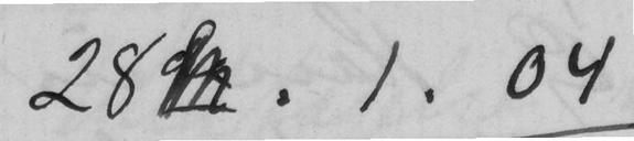28  .1.04
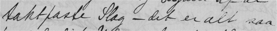taktfaste Slag - det er alt saa
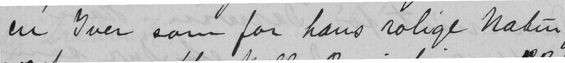en Iver som for hans rolige Natur
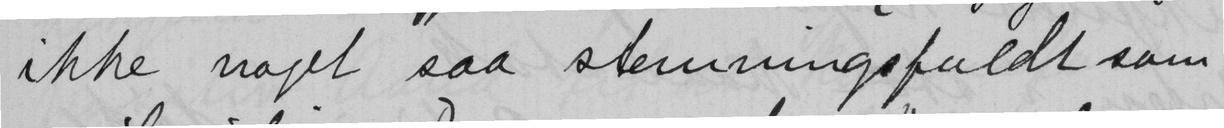ikke noget saa stemningsfuldt som
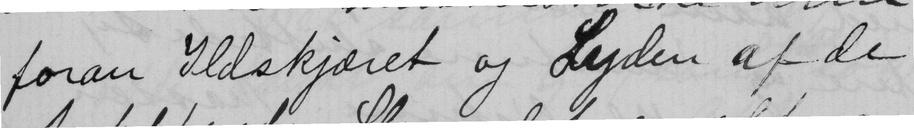foran Ildskjæret og Lyden af de
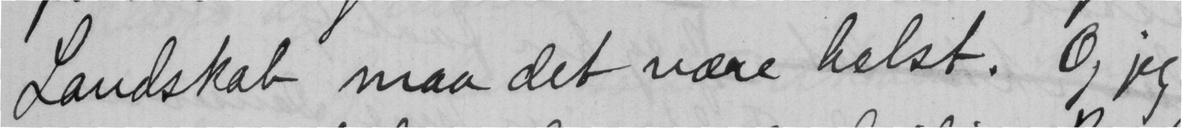Landskab maa det være helst. Og jeg
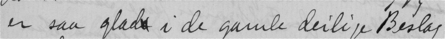er saa glad i de gamle deilige Beslag
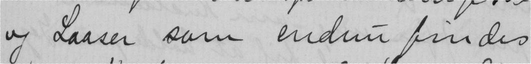og Laaser som endnu findes
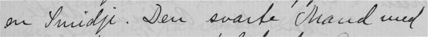en Smidje. Den svarte Mand med
